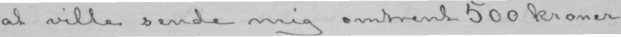at ville sende mig omtrent 500 kroner
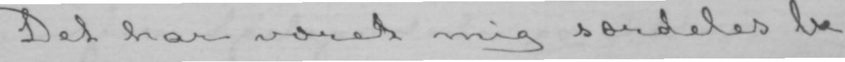Det har været mig særdeles be-
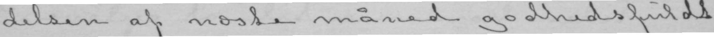delsen af næste måned godhedsfuldt
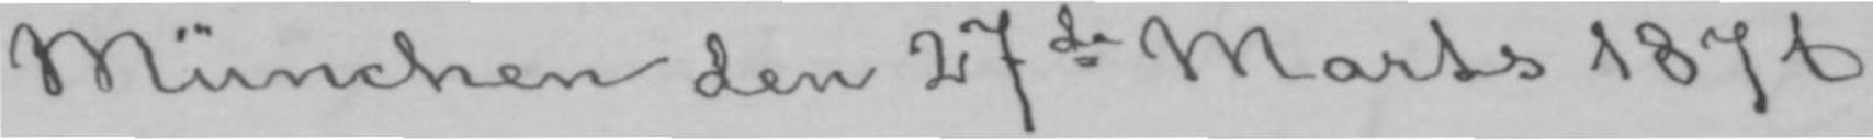München den 27de Marts 1876.
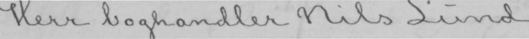Herr boghandler Nils Lund.
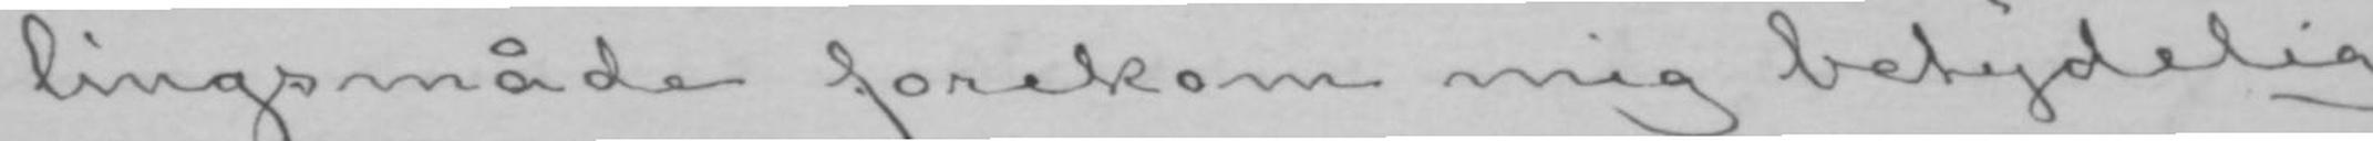lingsmåde forekom mig betydelig
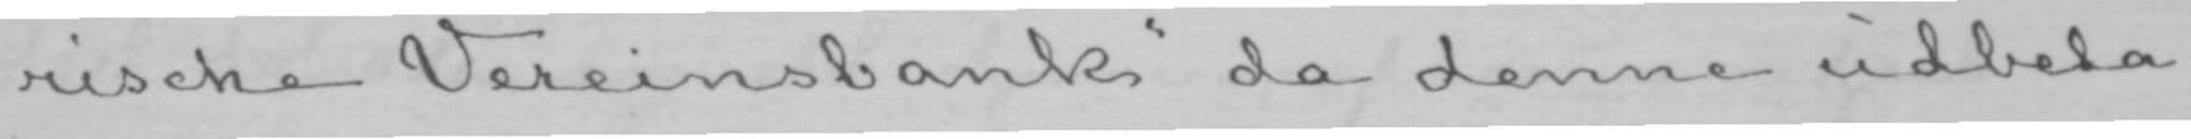rische Vereinsbank» da denne udbeta-
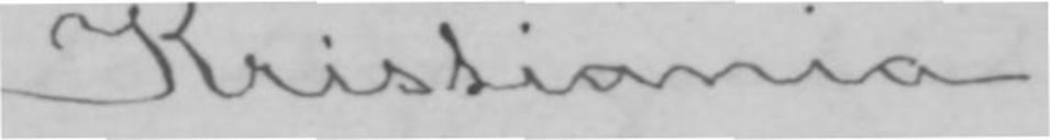Kristiania.
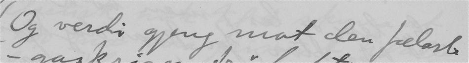Og verdi gjeng, mot den fælaste
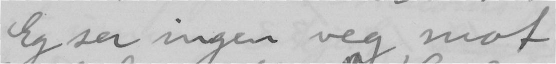Eg ser ingen veg mot
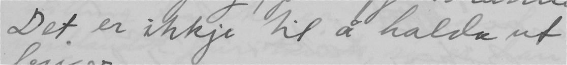Det er ikkje til å halde ut
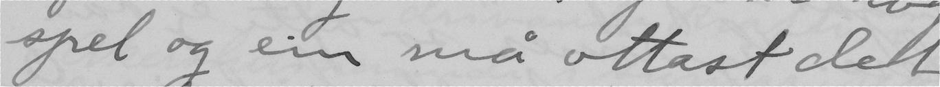spel og ein må ottast det
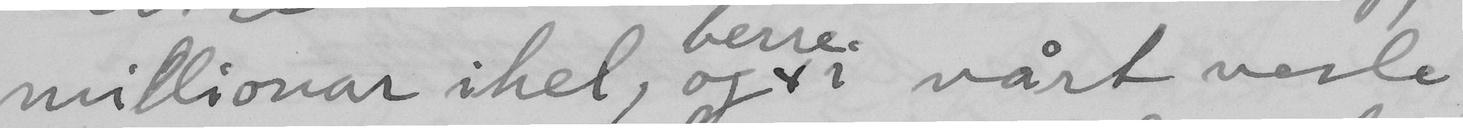millionar ihel, og berre i vårt vesle
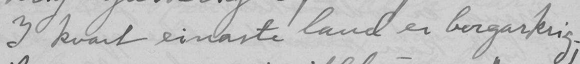I kvart einaste land er borgarkrig;
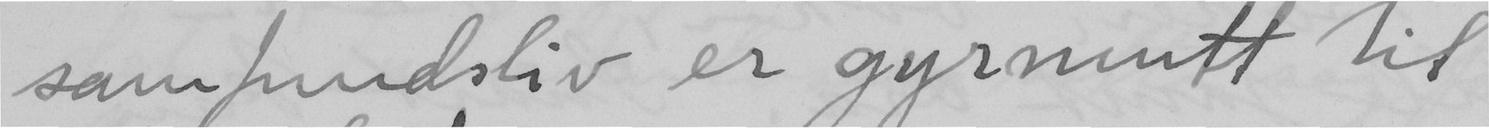samfundsliv er gyrmutt til
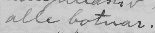alle botnar.
